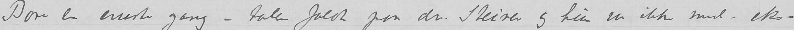Bare en eneste gang - talen faldt paa dr. Steiner og hun var ikke med - eks-
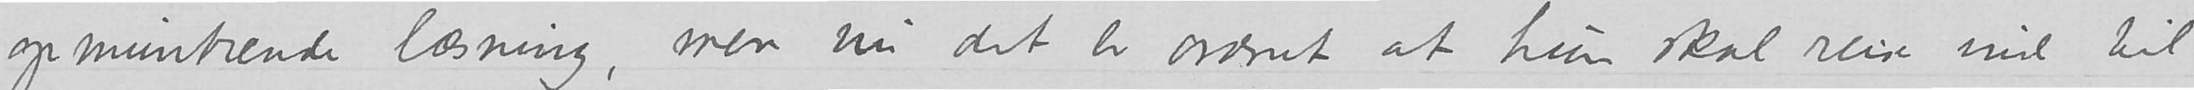opmuntrende læsning, men nu det er ordnet at hun skal reise ind til
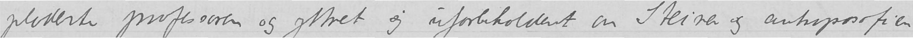ploderte professoren og yttret seg uforbeholdent om Steiner og antroposofien
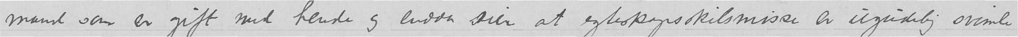mand som er gift med hende og endda sier at egteskapsskilsmisse er ugudelig svimle
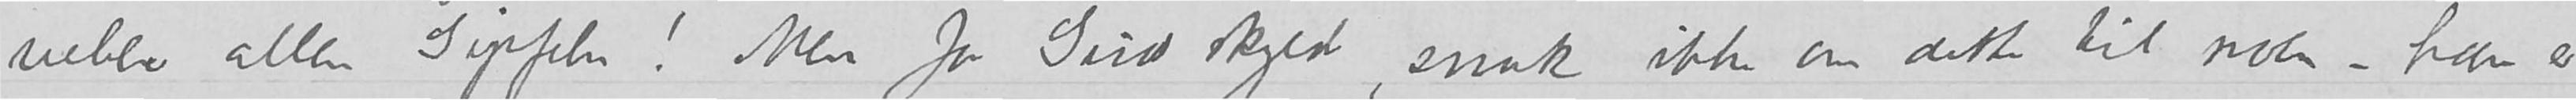svinde ueber allen Gipfeln! Men for Guds skyld, snak ikke om dette til noen - han er
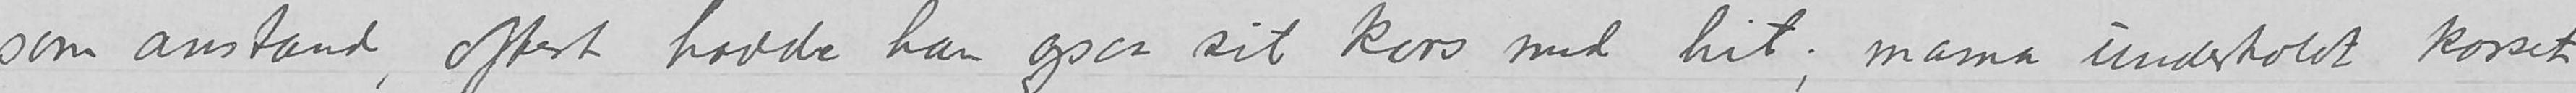som anstand, oftest hadde han ogsaa sit kors med hit; mama underholdt korset
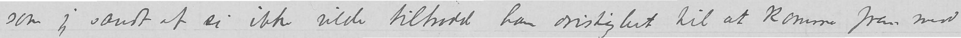som jeg sandt at si ikke vilde tiltrodd ham dristighet til at komme fram med
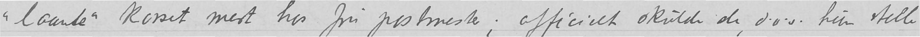laante korset mest hos fru postmester; officielt skulde de, d.v.s. hun stelle
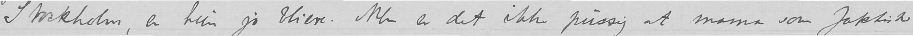Stockholm, er hun jo bliere. Men er det ikke pussig at mama som faktisk
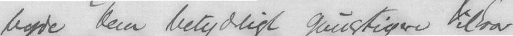byde Dem betydeligt gunstigere tilsvar
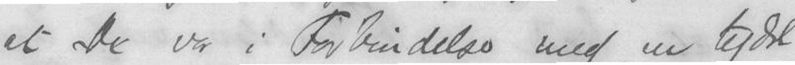at De var i Forbindelse med en tydsk
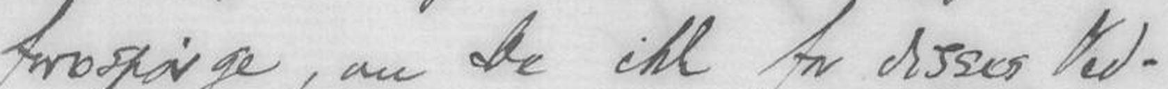forspørge, om De ikke for disses Ved-
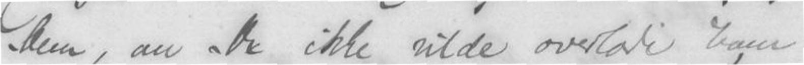Dem, om De ikke vilde overlade ham
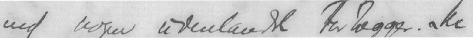end nogen udenlands Forlægger. De
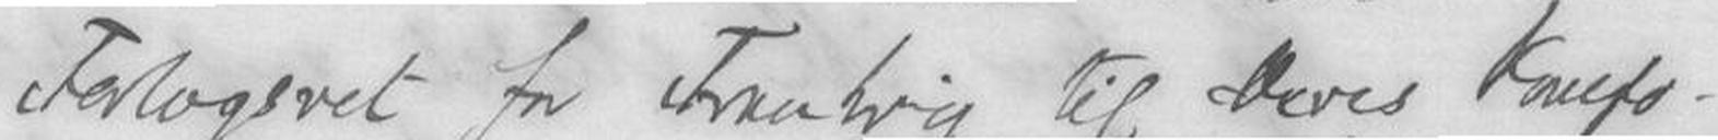Forlagsret for Frankrig til deres Kompo-
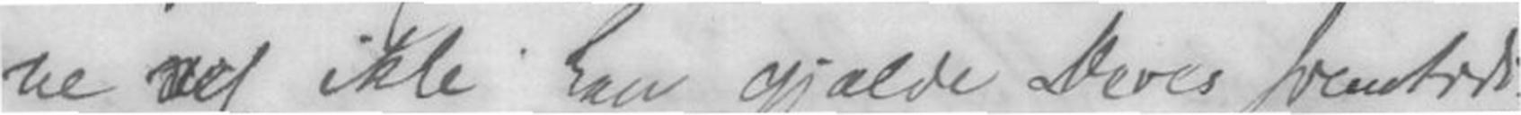ne vel ikke kan gjælde Deres fremtidi-
